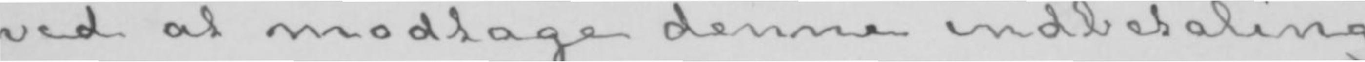ved at modtage denne indbetaling
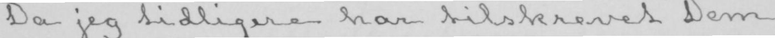Da jeg tidligere har tilskrevet Dem
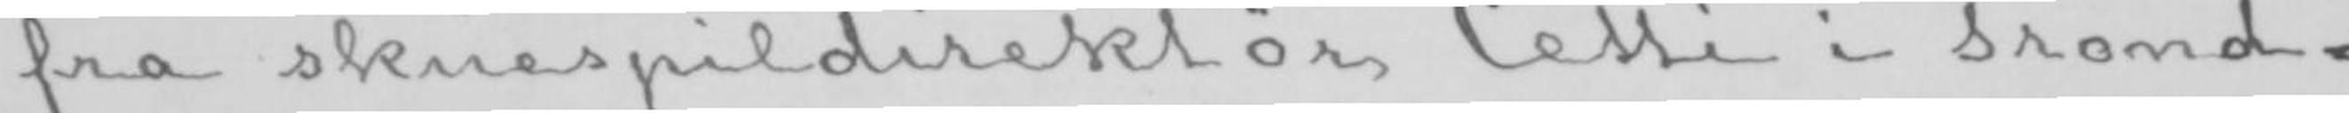fra skuespildirektør Cetti i Trond-
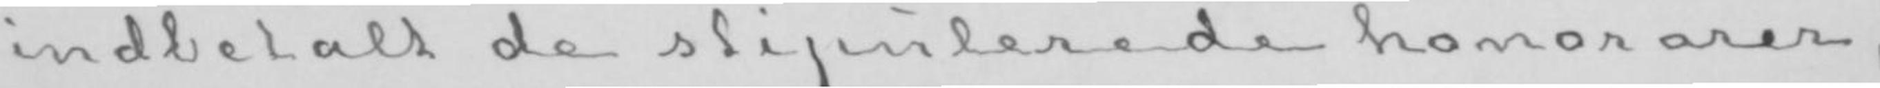indbetalt de stipulerede honorarer,
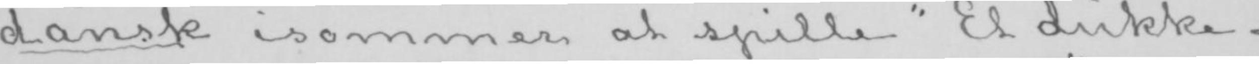dansk isommer at spille «Et dukke-
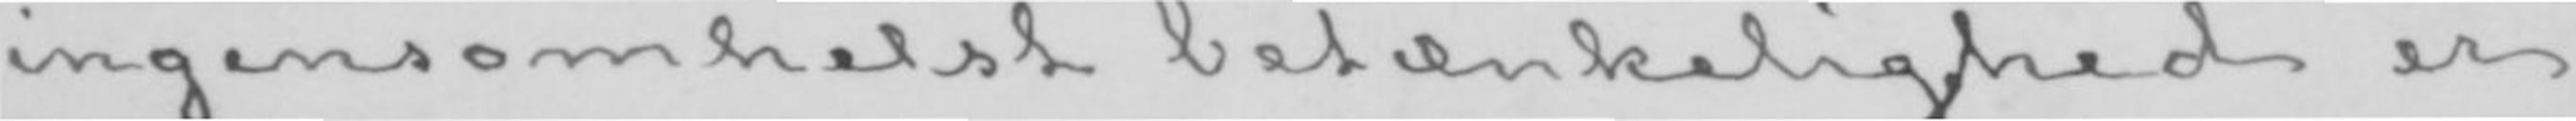ingensomhelst betænkelighed er
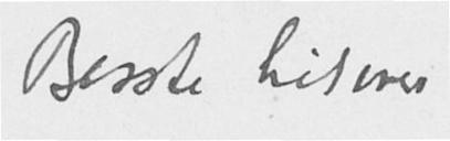Besste hilsener
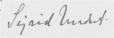Sigrid Undset.
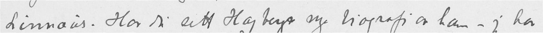Linnæus. Har du sett Hagbergs nye biografi om ham – jeg har
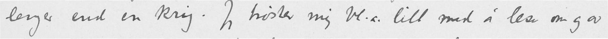lenger end en krig. Jeg trøster mig bl.a. litt med å lese om og av
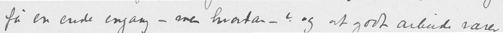få en ende engang – men hvordan –? og at godt arbeide varer
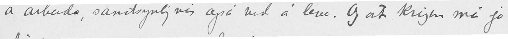å arbeide, sandsynligvis også ved å leve. Og at krigen må jo
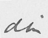din
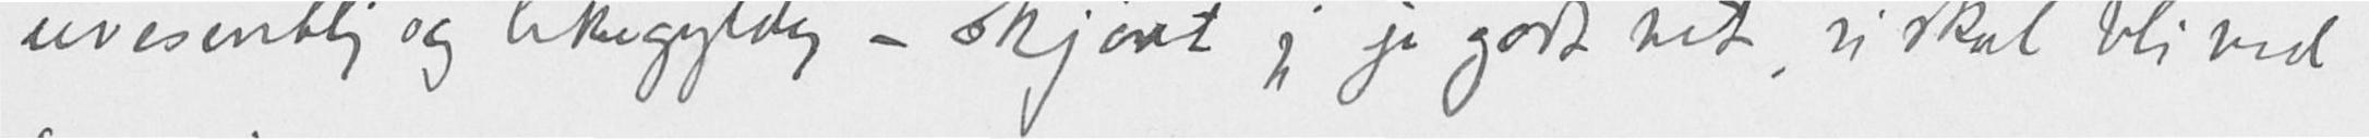uvesentlig og likegyldig - skjønt jeg jo godt vet, vi skal bli ved
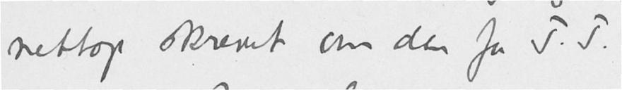nettop skrevet om den for T.T.
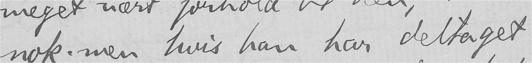nok; men hvis han har deltaget
